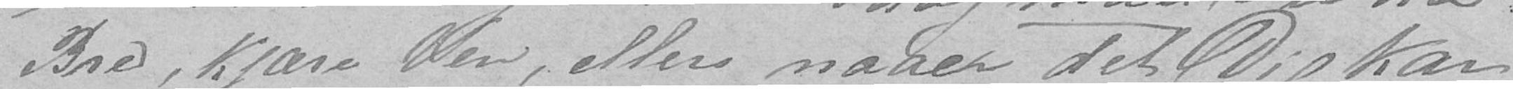Brev, Kjære Ven, ellers naaer det Dig kan-
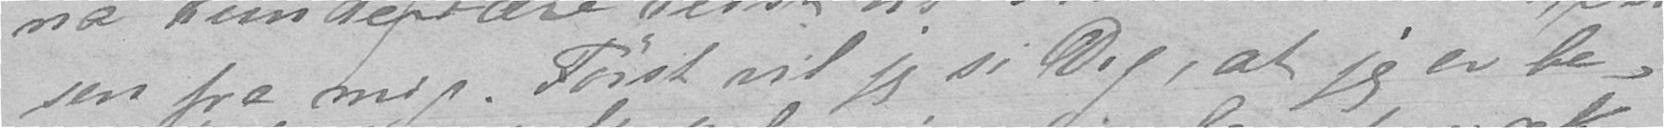sen fra mig. Først vil jeg si Dig, at jeg er be-
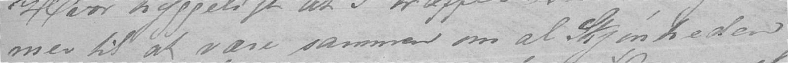mer til at være sammen om al Skjønheden
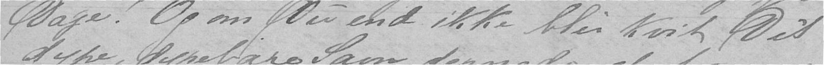Dage. Og om Du end ikke blir kvit Dit
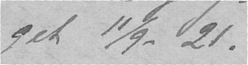get 11/9- 21.
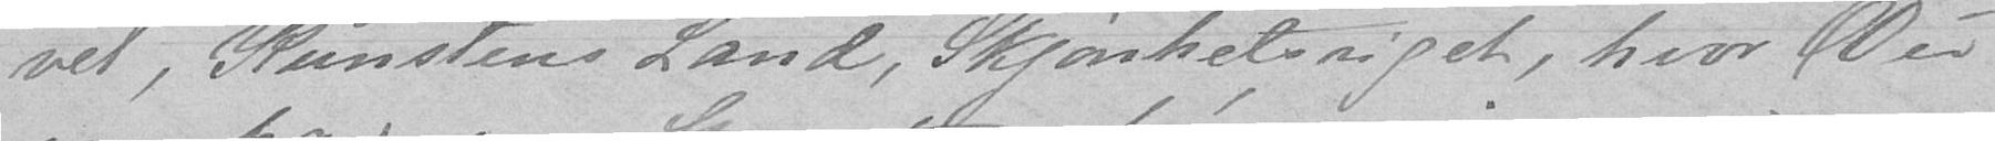vel, Kunstens Land, Skjønhetsriget, hvor Du
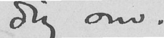dig om.
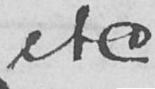etc.
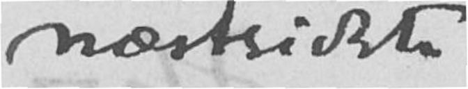næstsidste
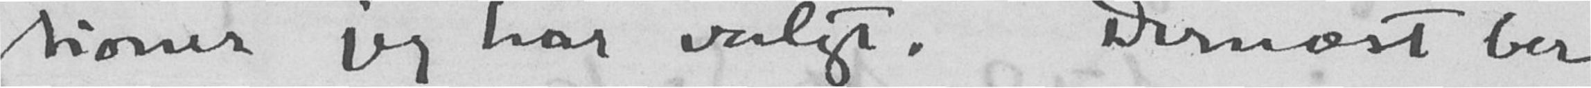tioner jeg har valgt. Dernæst ber
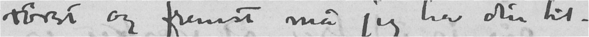Først og fremst må jeg ha din til-
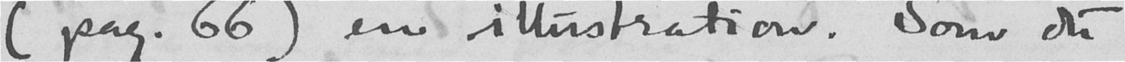(pag. 66) en illustration. Som du
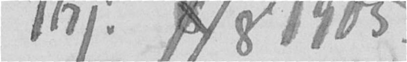Thj. 7/8 1905.
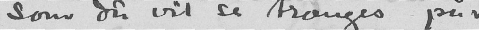Som du vil se trænges på
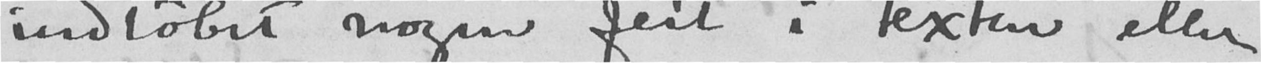indløbet nogen feil i texten eller
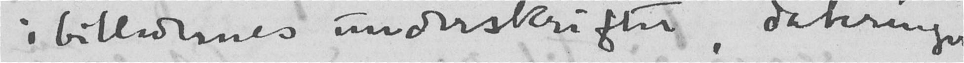i billedernes underskrifter, dateringer
